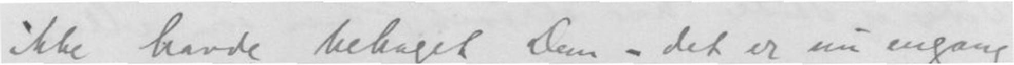ikke havde belaget Dem - det er du engang
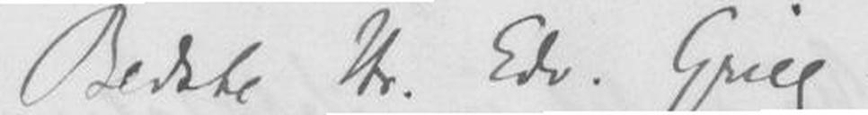Beste Hr. Ed. Grieg
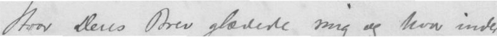Hvor Deres Brev glædede mig og hvor inder-
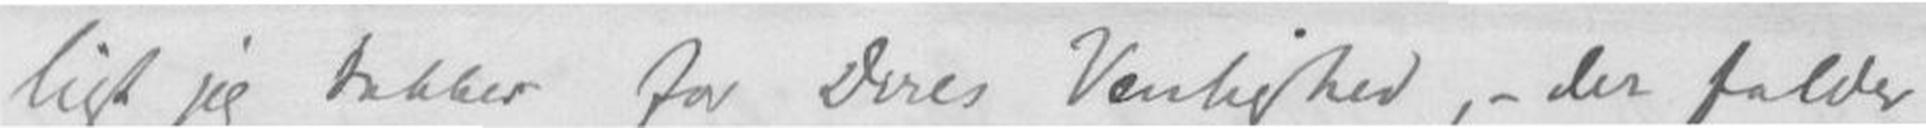ligt jeg takker for venlighed,. der falder
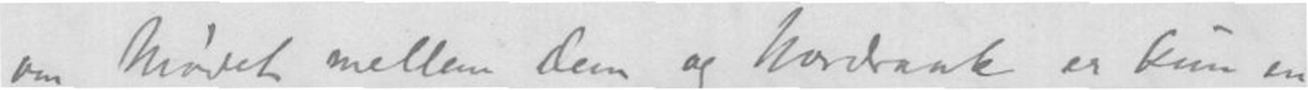om Møtet mellom Dem og Nordaak er kun en
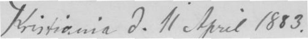Kristiania D.1 April 1883
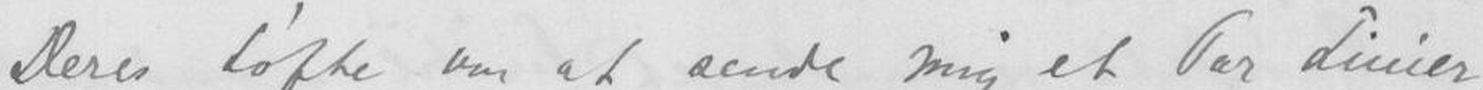Deres løfte om at sende mig et Var Linier,
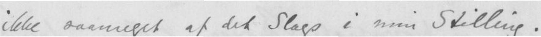ikke saameget af det Slags i min Stilling.
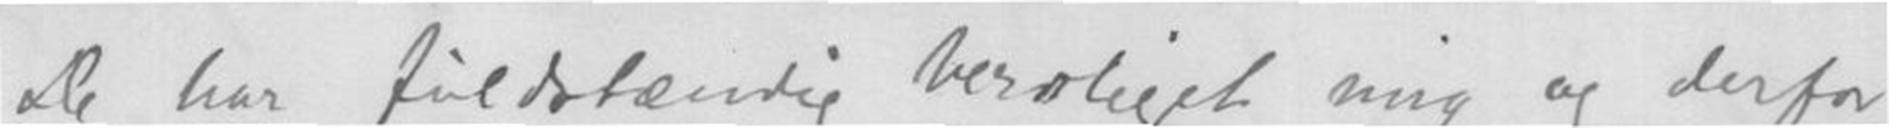De har fulstændig beroliget mig og derfor
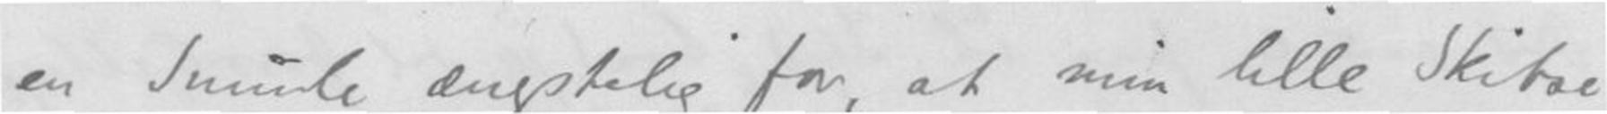en Smule ængstelig for, at min lille Skitse
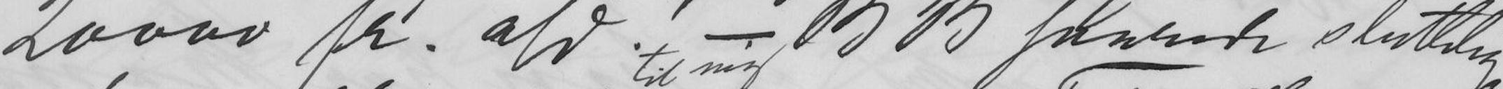20000 fr osv. - B B svarede sluttelig
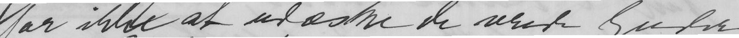for ikke at udæske de vrede Guder
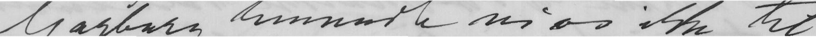Garborg henvendte vi os ikke til
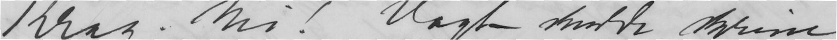Krag. Nei! Uagt skulde skrive
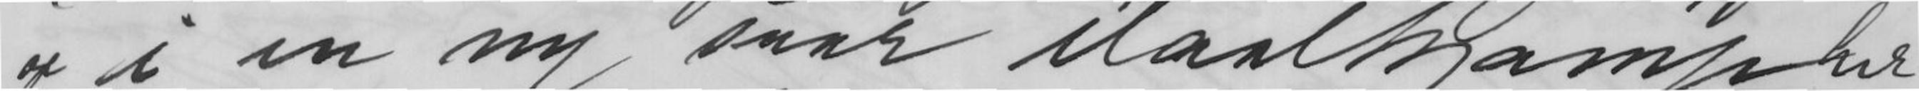op i en ny saar Maalkamp her.
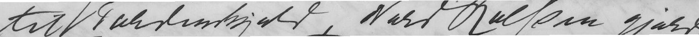til Tordenskjold, Nord Rolfsen gjorde
